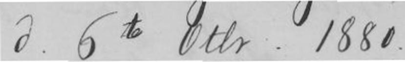d. 6te Otbr. 1880.
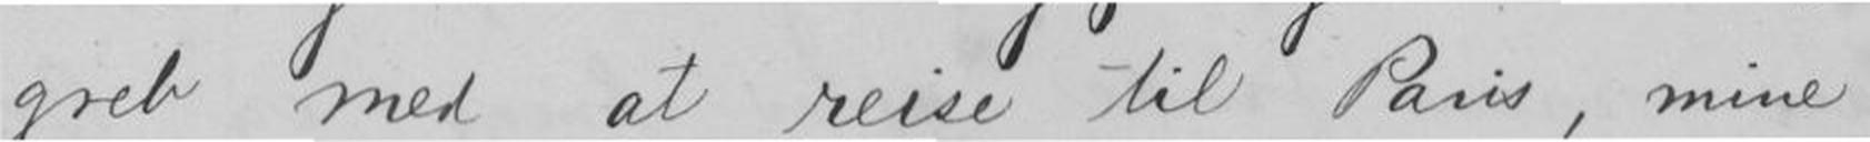greb med at reise til Paris, mine
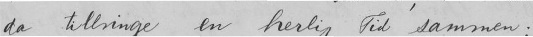da tilbringe en herlig Tid sammen;
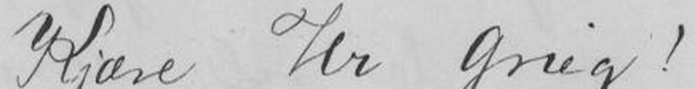Kjære Hr. Grieg!
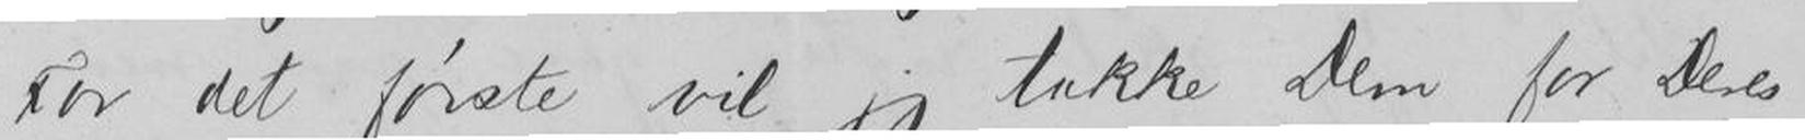For det første vil jeg takke Dem for Deres
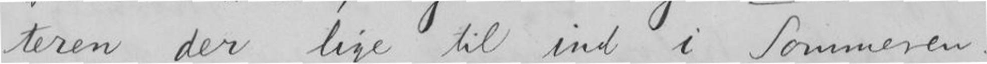teren der lige til ind i Sommeren!
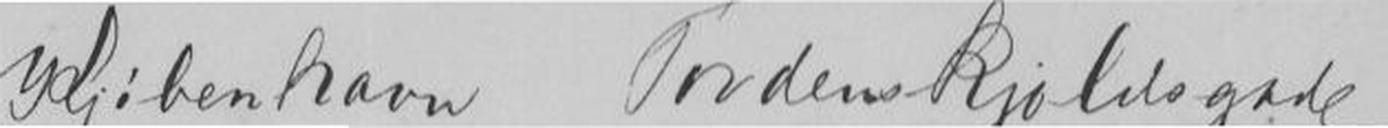Kjøbenhavn Tordenskjøldsgade 1
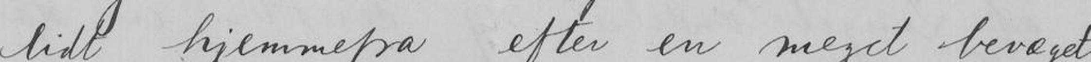lidt hjemmefra efter en meget bevæget
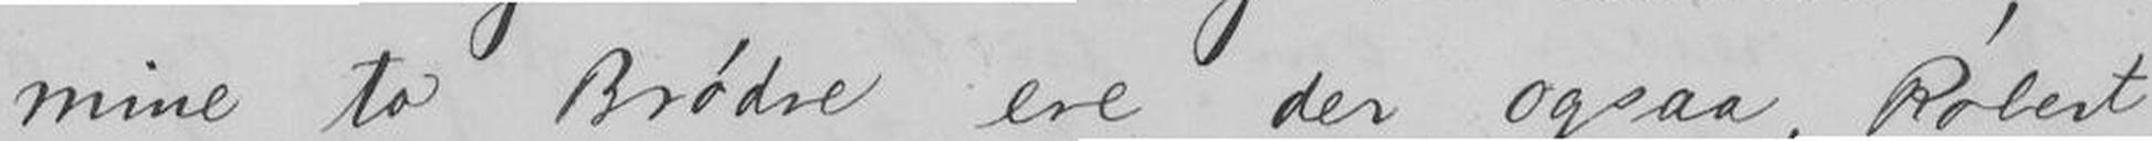mine to Brødre ere der ogsaa, Robert
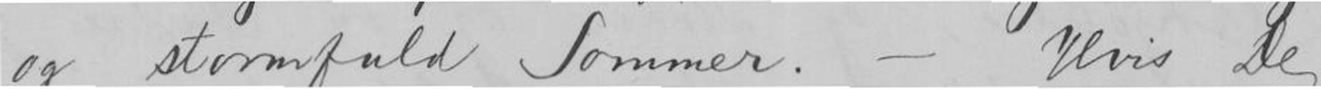og stormfuld Sommer.- Hvis De
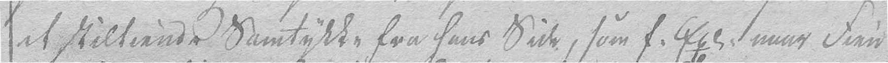et stiltiende Samtykke fra hans Side, som f: Exl: naar Fien-
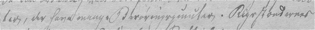ter, der have mange Berøringspuncter. Rigsstændernes
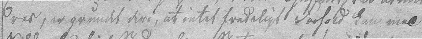res, er grundet deri, at intet frædeligt Forhold kan med
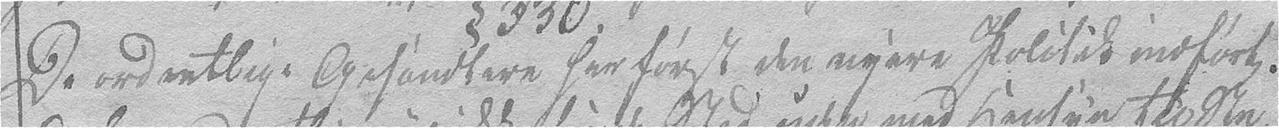De ordentlige Gesandtere har først den nyere Politik indført.
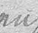en
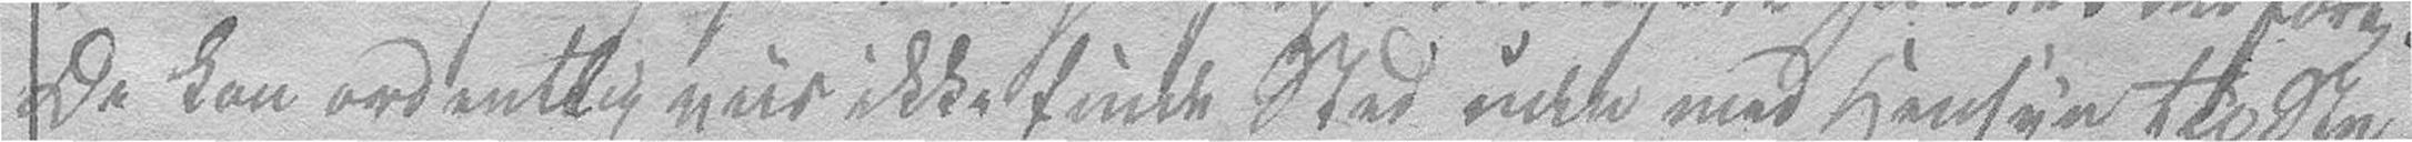De kan ordentlig viis ikke finde Sted uden med Hensyn til Sta-
